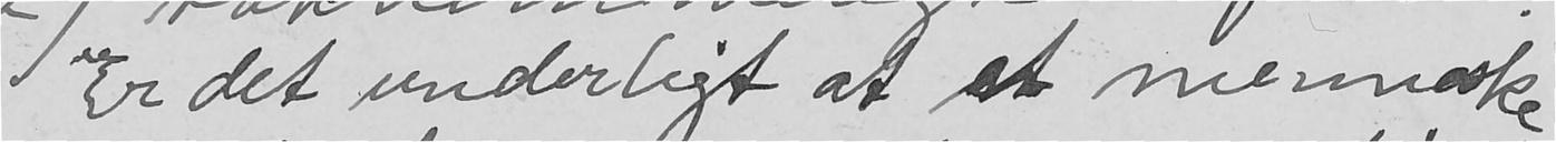Er det underligt at et menneske
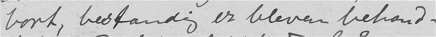bort, bestandig er bleven behand-
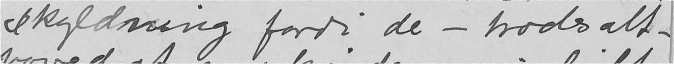skyldning fordi de - trods alt -
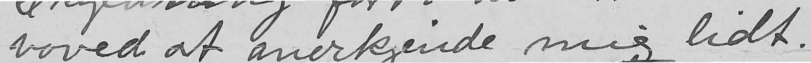voved at anerkjende mig lidt.
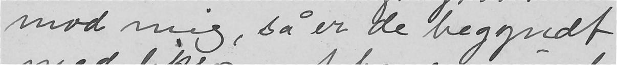mod mig, så er de begyndt
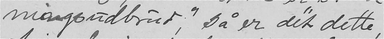mningsudbrud", så er det dette,
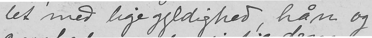let med ligegyldighed, hån og
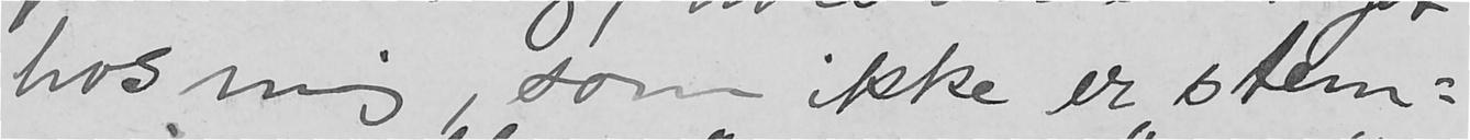hos mig, som ikke er "stem-
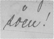søen!
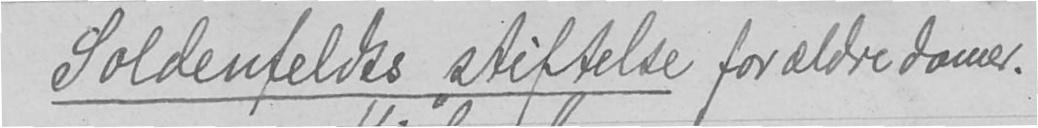Soldenfelts stittelse for ældre damer.
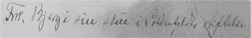Frk. Bjerg i sin stue i Soldefelts stiftelse.
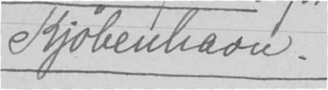Kjøbenhavn.
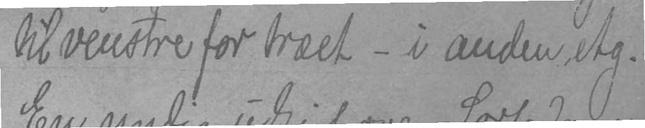til venstre for træet - i anden etg.
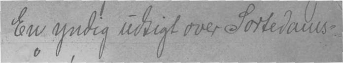En yndig udsigt over Sortedams-
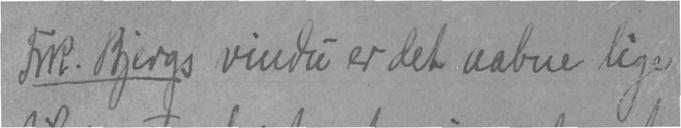Frk. Bjergs vindu er det aabne lige
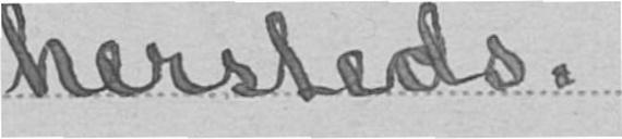hersteds.
